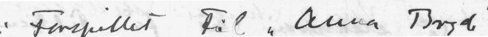i Forspillet tilAnna Bryde
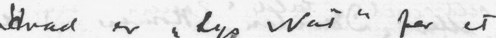Hvad er "Lys Nat" for et
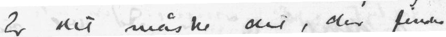Er det måske det, der finder
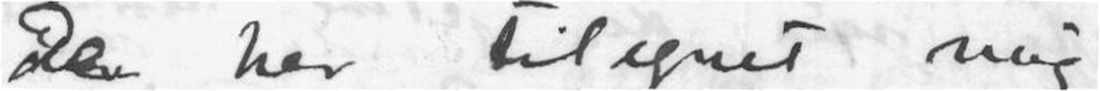De har tilegnet mig.
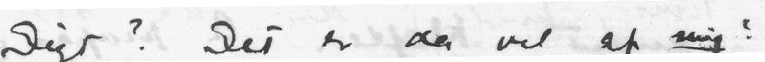Digt? Det er da vel af mig?
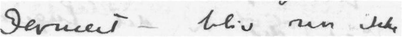Dermest - bliv nu ikke
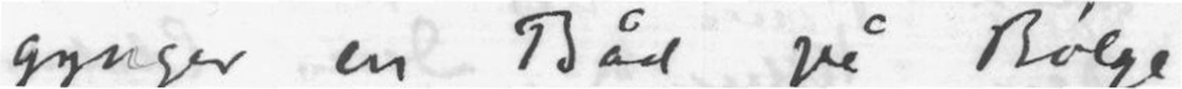gynger en Båd på Bølge
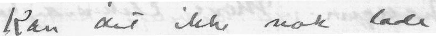Kan det ikke nok lade
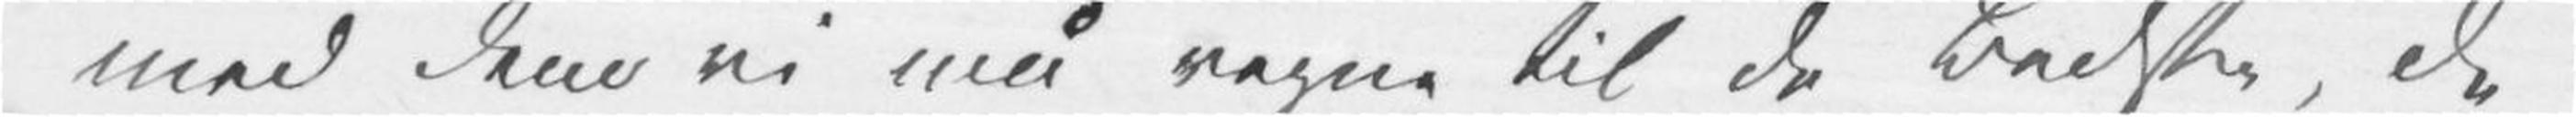med dem vi må regne til de Bedste, de
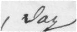dog
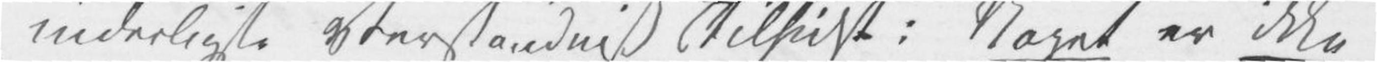inderligste «Verständniss» tilsidst: Noget er ikke
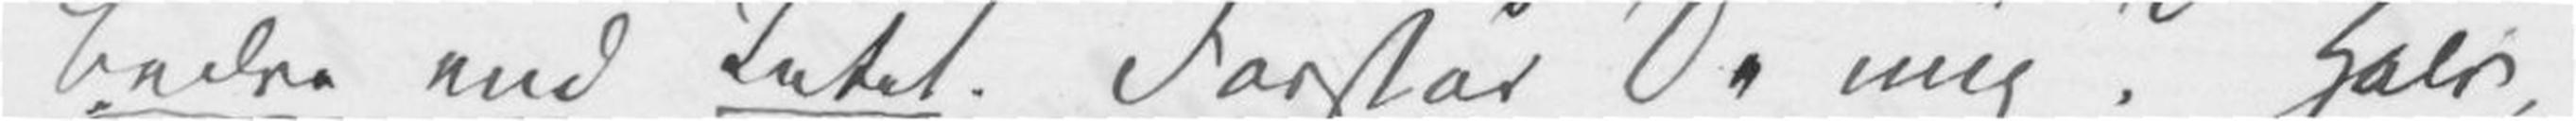bedre end Intet. Forstår De mig? Halv,
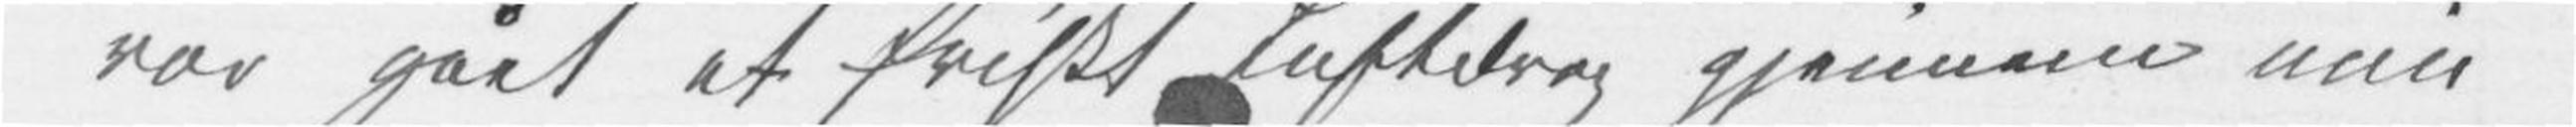var gået et friskt Luftdrag gjennem min
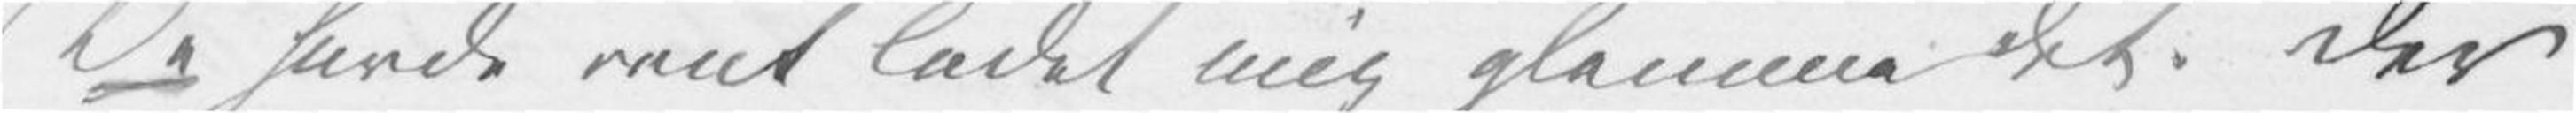De havde rent ladet mig glemme det. Der
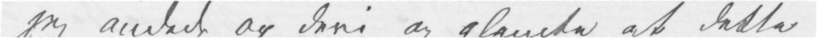jeg åndede op deri og glemte at dette
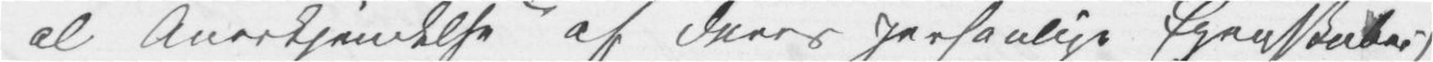al Anerkjendelse af deres personlige Egenskaber)
